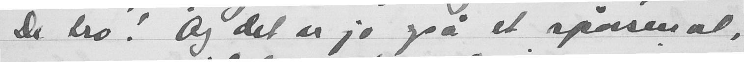De tro! Og det er jo også et spørsmål,
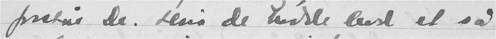forstår De. Hvis de haddle levd et så
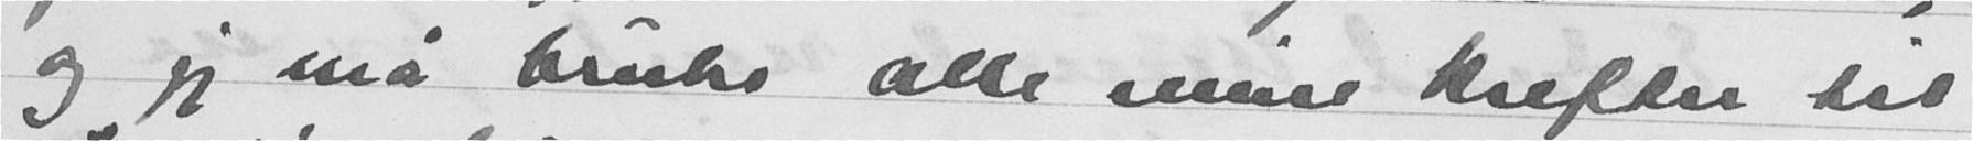og jeg må bruke alle mine krefter til
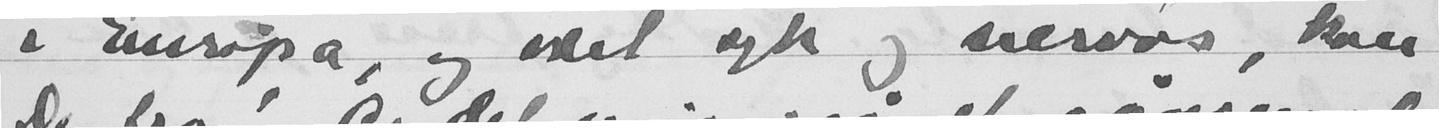i Europa, og vært syk og nervøs, kan
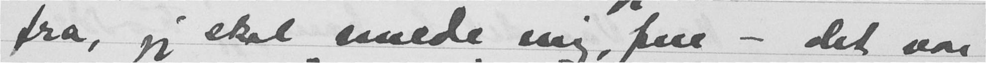fra, jeg skal melde mig frue - det var
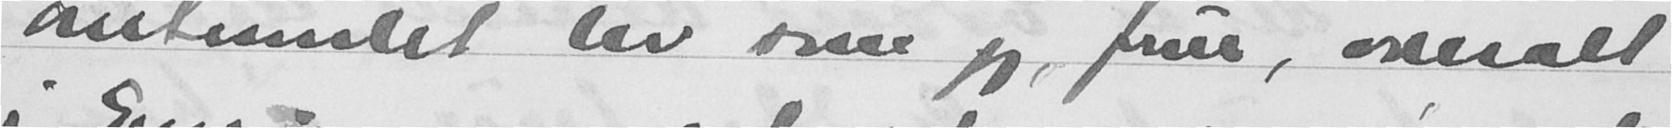omtumlet liv som jeg frue, overalt
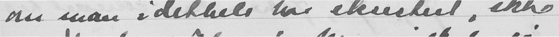om man i dethele har eksistert, ikke
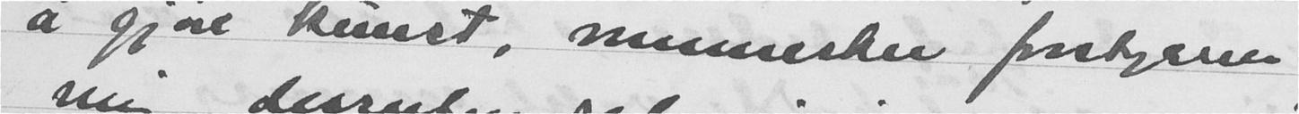å gjøre kunst, mennesker forstyrrer
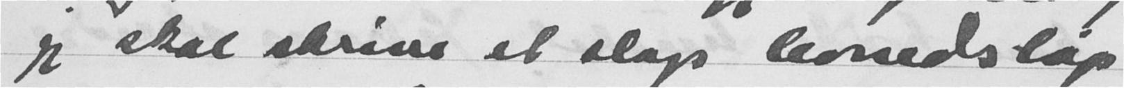jeg skal skrive et slags levnedslap
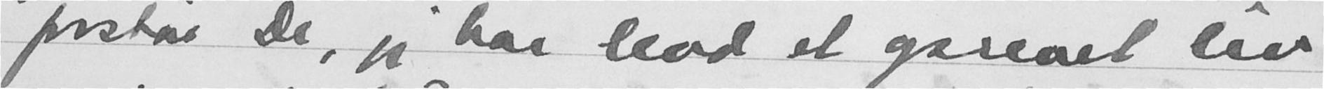forstår De, jeg har levd et oprevet liv
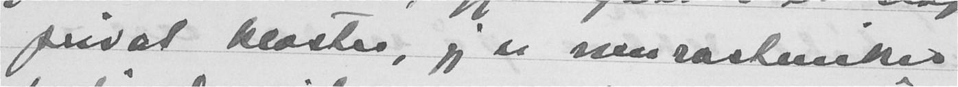privat kloster, jeg er nevrasteniker
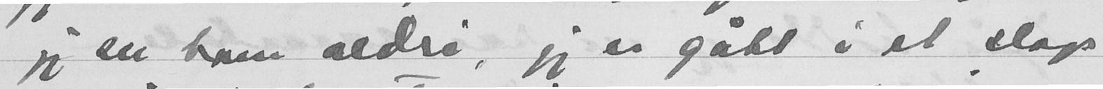jeg ser ham aldri, jeg er gått i et slags
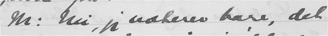M: Nu jeg noterer bare, det
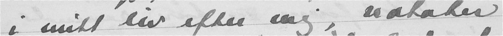i mitt liv efter mig, notater
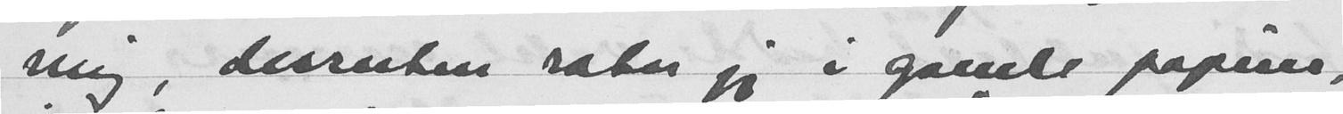mig, dessuten roter jeg i gamle papirer,
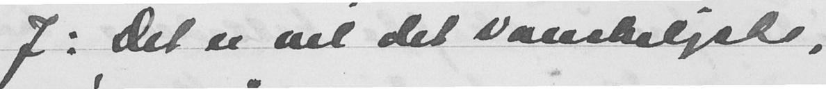J: Det er vel det vanskeligste,
